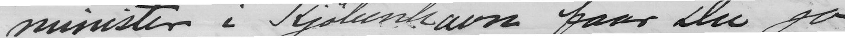minister i Kjøbenhavn faar Du jo
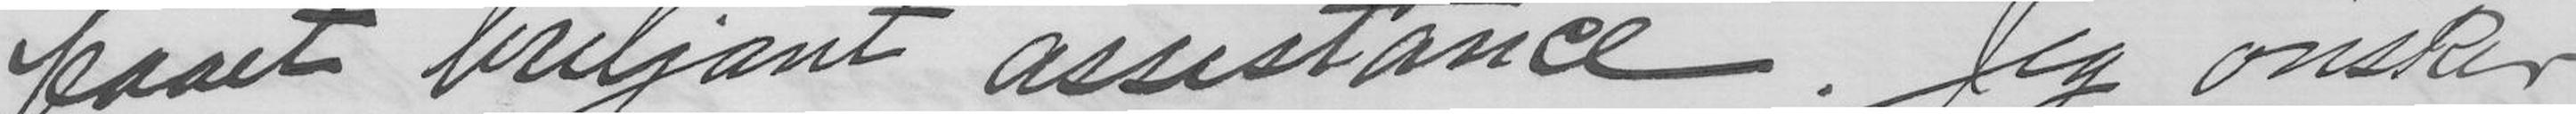faaet briljant assistance. Jeg ønsker
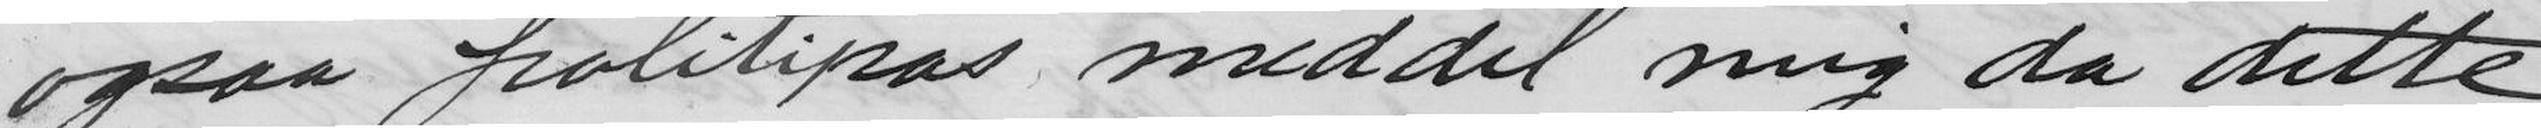ogsaa politipas meddel mig da dette
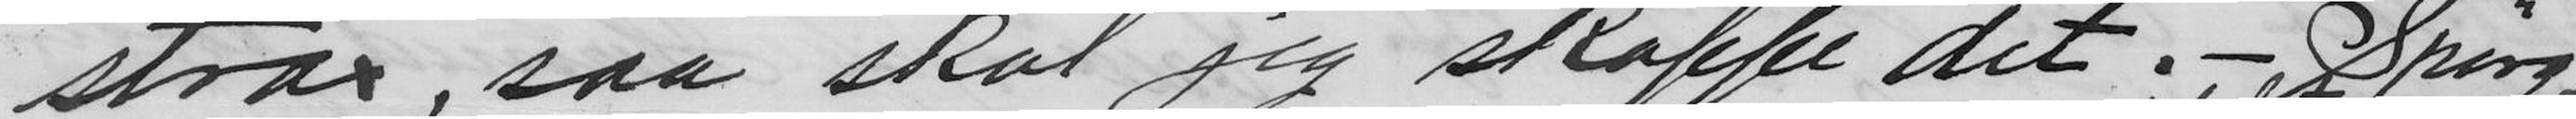strax, saa skal jeg skaffe det. - Spørg
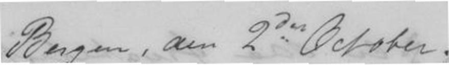Bergen, den 2den October.
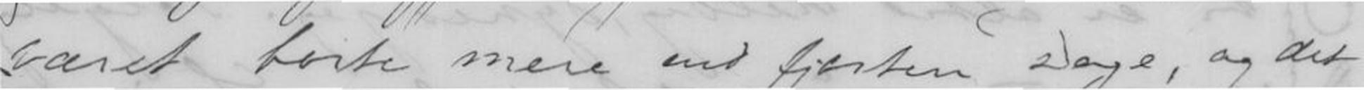været borte mere end fjorten Dage, og det
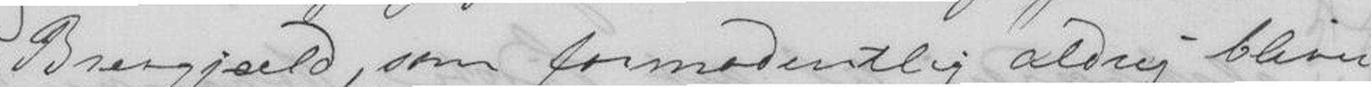Brevgjeld, som formodentlig aldrig bliver
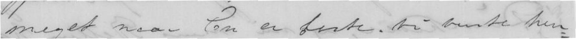meget naar En er borte; vi vente hen-
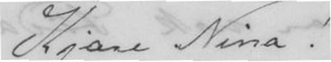Kjære Nina!
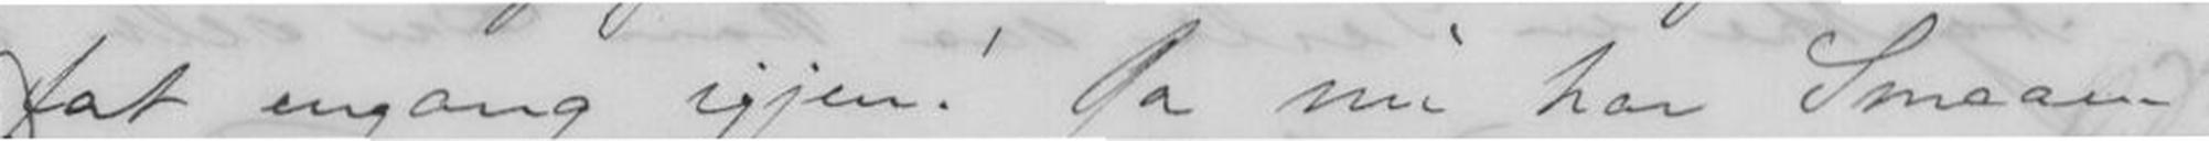fat engang igjen! Ja nu har Smaaen
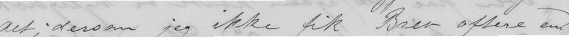det; dersom jeg ikke fik Brev oftere end
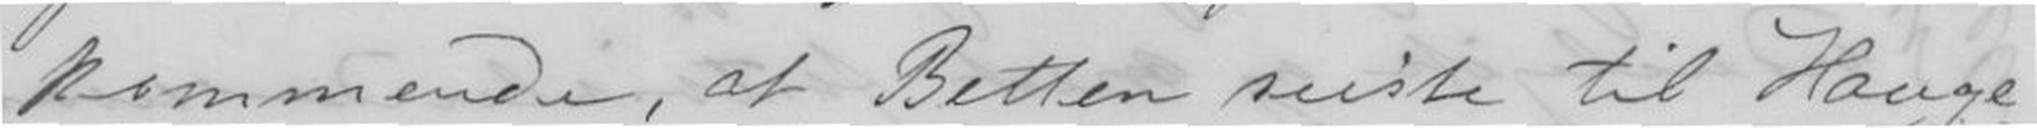kommende, at Betten reiste til Hauge-
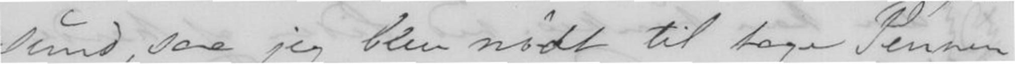sund, saa jeg blev nødt til tage Pennen
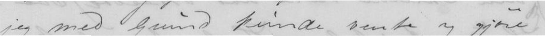jeg med Grund kunde vente og gjøre
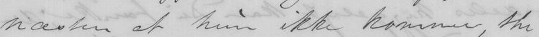næsten at hun ikke komme, thi
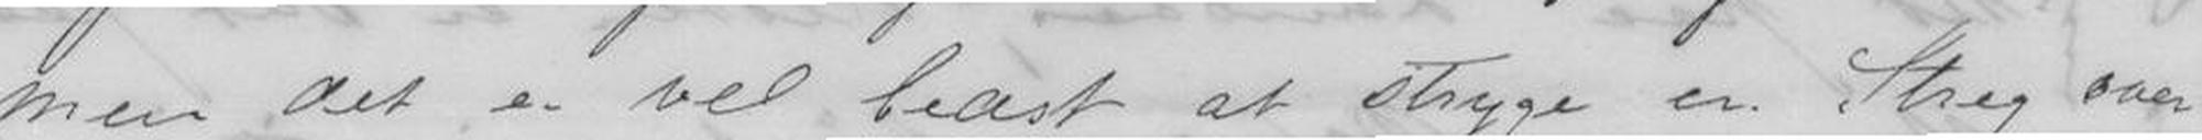men det er vel bedst at stryge en Streg over
